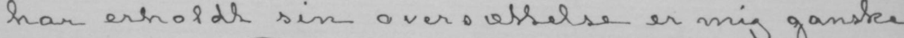har erholdt sin oversættelse er mig ganske
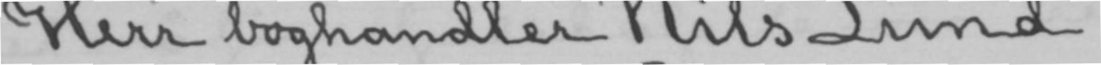Herr boghandler Nils Lund.
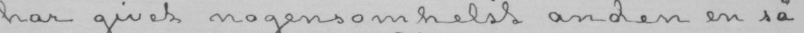har givet nogensomhelst anden en så-
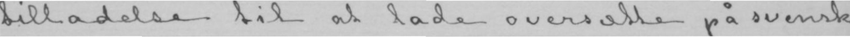tilladelse til at lade oversætte på svensk
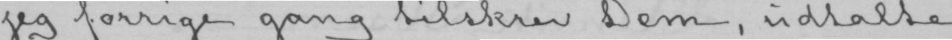jeg forrige gang tilskrev Dem, udtalte
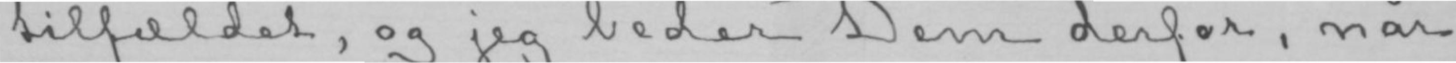tilfældet, og jeg beder Dem derfor, når
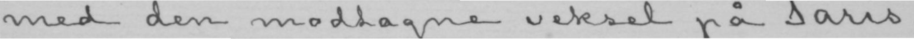med den modtagne veksel på Paris.
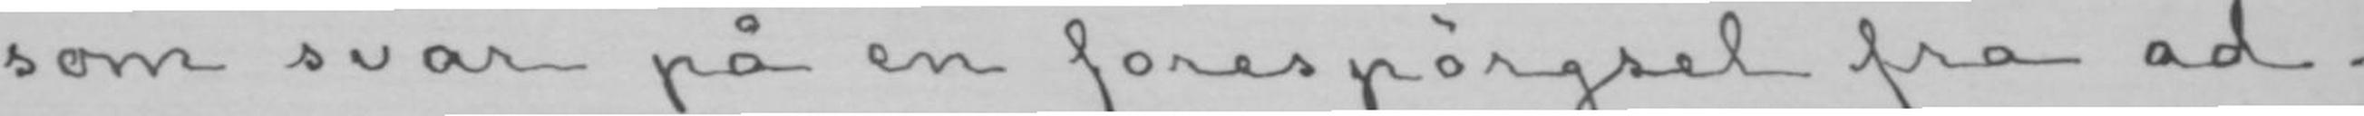som svar på en forespørgsel fra ad-
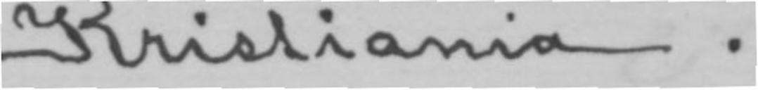Kristiania.
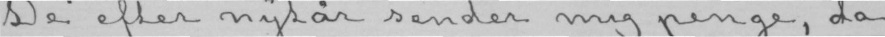De efter nytår sender mig penge, da
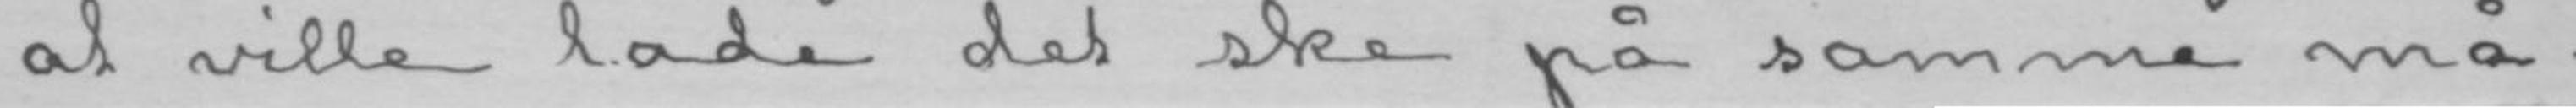at ville lade det ske på samme må-
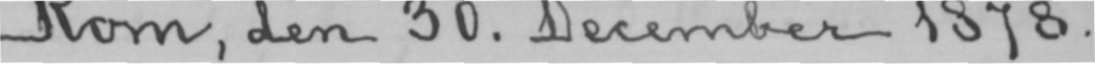Rom, den 30. December 1878.
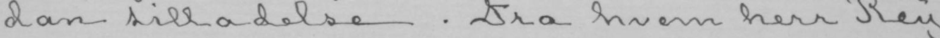dan tilladelse. Fra hvem herr Key
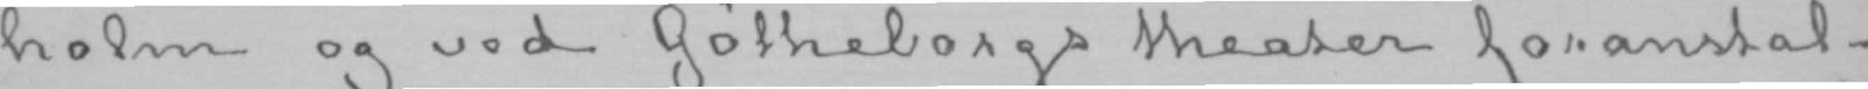holm og ved Götheborgs theater foranstal-
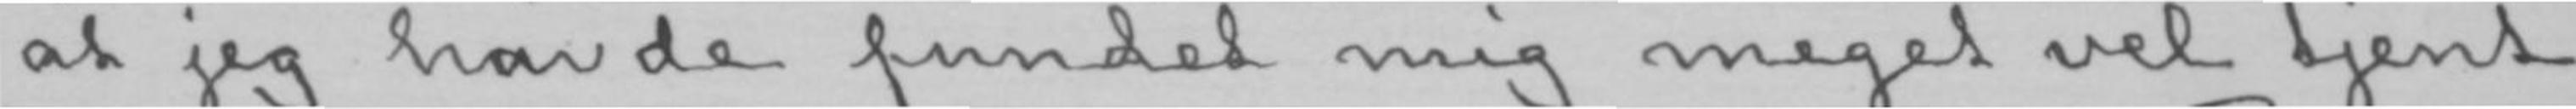at jeg havde fundet mig meget vel tjent
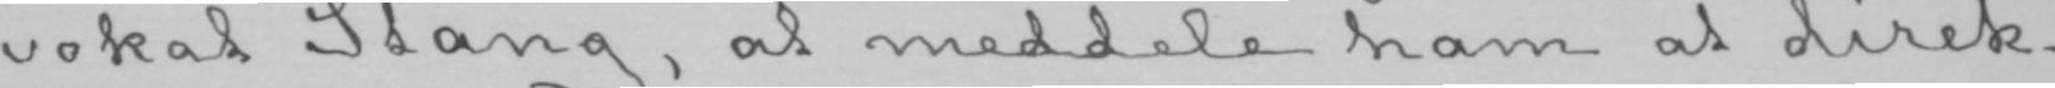vokat Stang, at meddele ham at direk-
